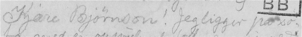Kjære Bjørnson! Jeg ligger på so-
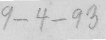9 - 4 - 93
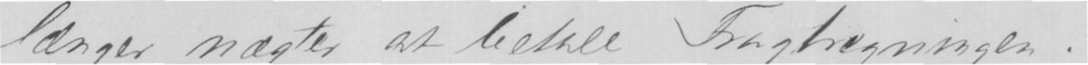længer nægter at betale Fragtregningen.-
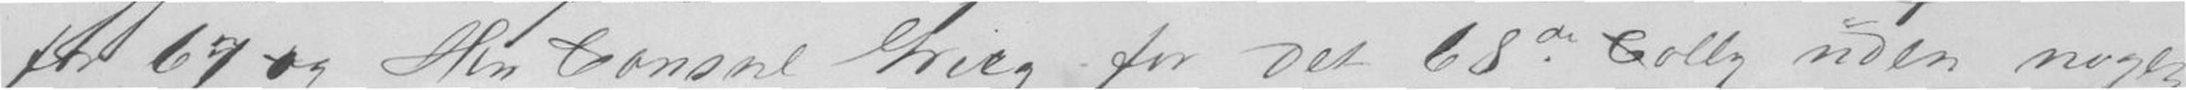for 67 og Herr Consul Grieg for det 68de Colly uden nogle
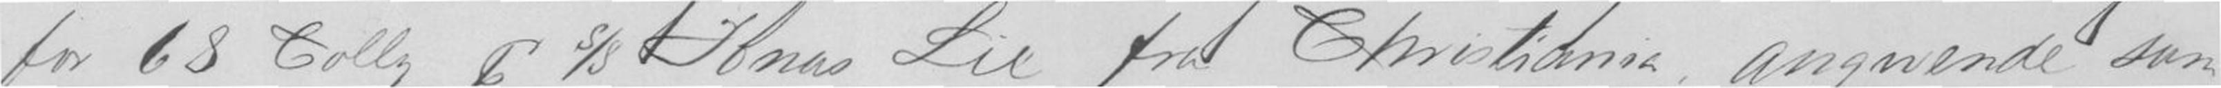for 68 Colly s/s Tomas Lie fra Christiania, angivende som
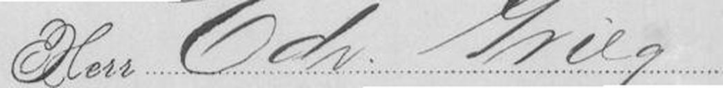Herr Edv. Grieg
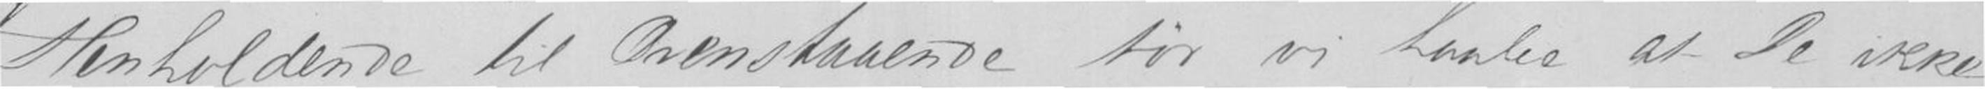Henholdende til Ovenstaaende tør vi haabe at De ikke
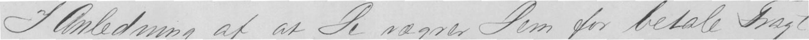I anledning af at De vægrer Dem for betale Fragt
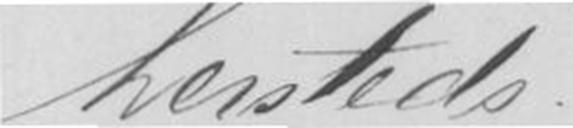hersteds.
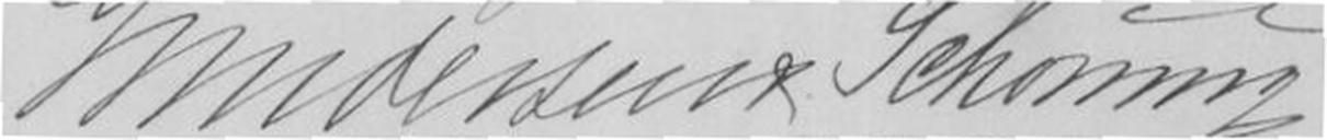Gundersen & Schøning
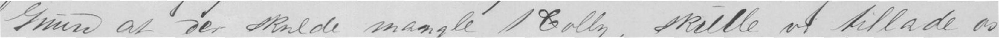Grund at det skulde mangle 1 Colly, skulle vi tillade os
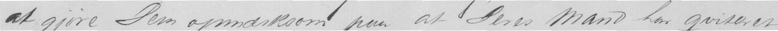at gjøre Dem opmærksom paa at Deres Mand har quitert
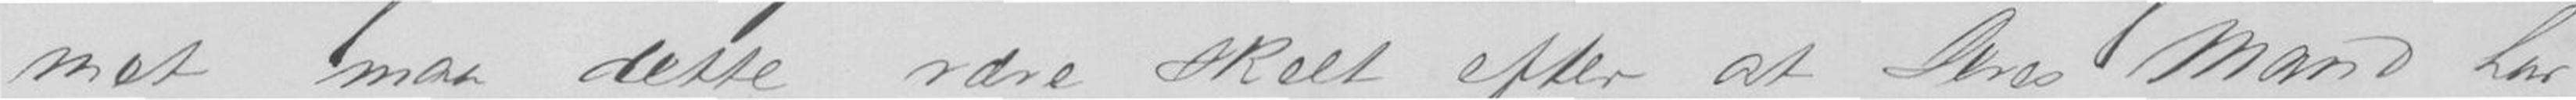met maa dette være skeet efter at Deres Mand har
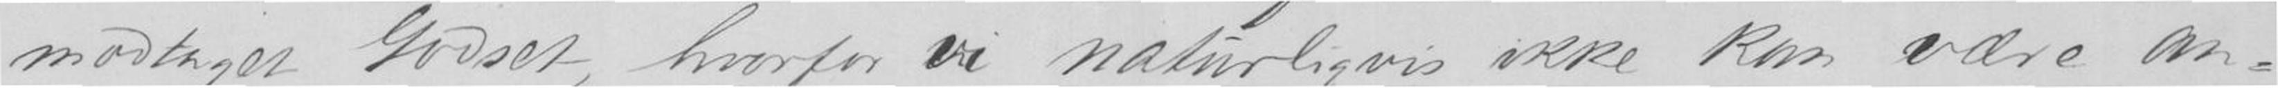modtaget Godset, hvorpaa vi naturligvis ikke kan være an-
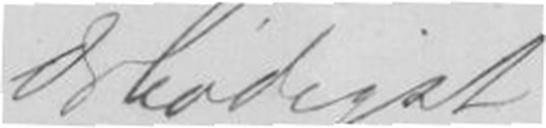Ærbødigst
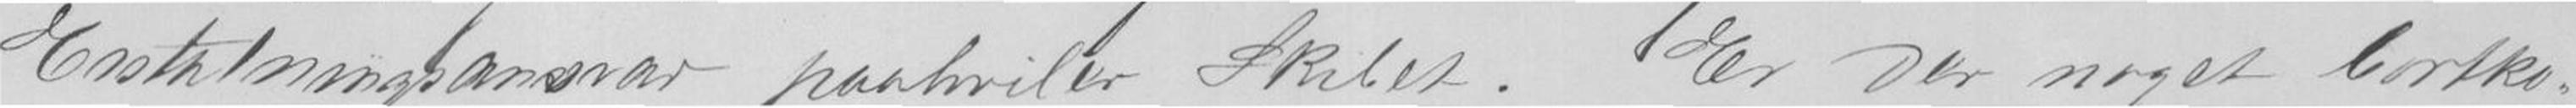Erstatningsansvar paahviler Skibet. Er der noget bortko-
